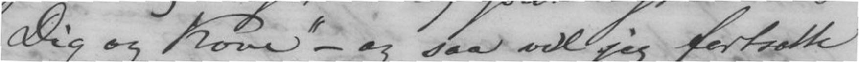Dig og Kone" - og saa vil jeg fortsætte
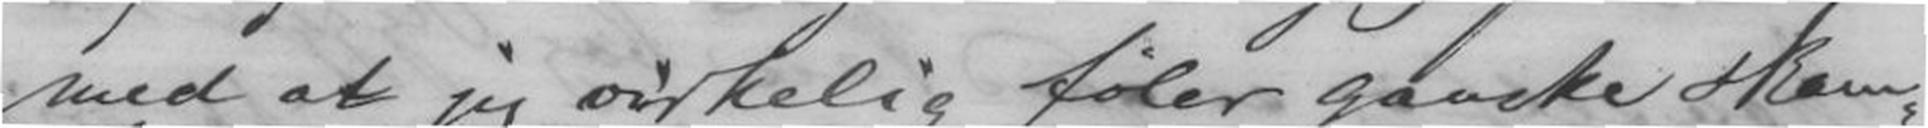med at jeg virkelig føler ganske skam-
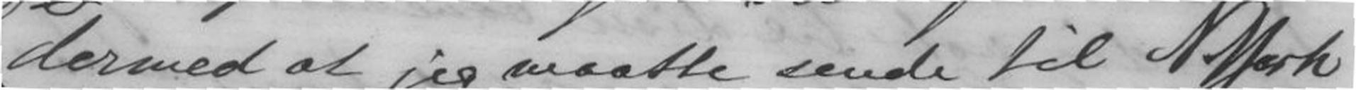dermed at jeg maatte sende til N. York
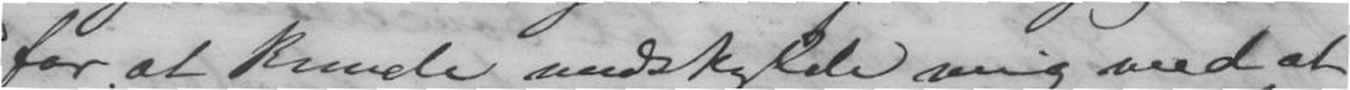for at kunde undskylde mig med at
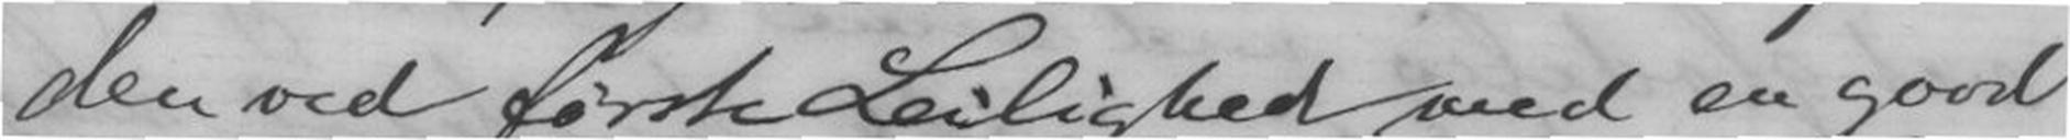den ved første Leilighed ved en good
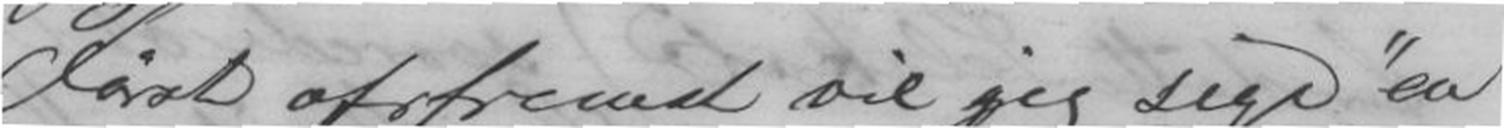Først af fremst vil jeg sige "en
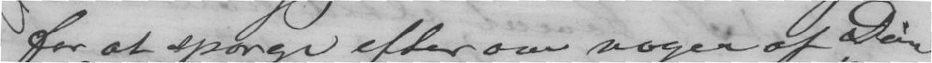for at spørge efter om nogen af Dine
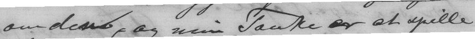om den, og min Tanke er at spille
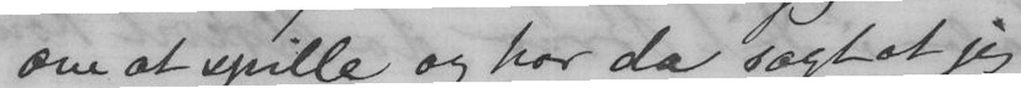om at spille og har da sagt at jeg
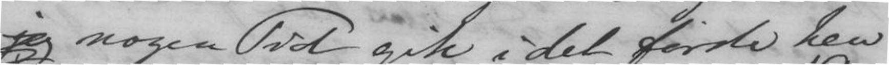nogen Tid gik i det føste hen
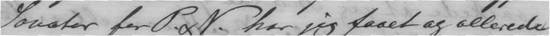Sonater for P & N. har jeg faaet og allerede
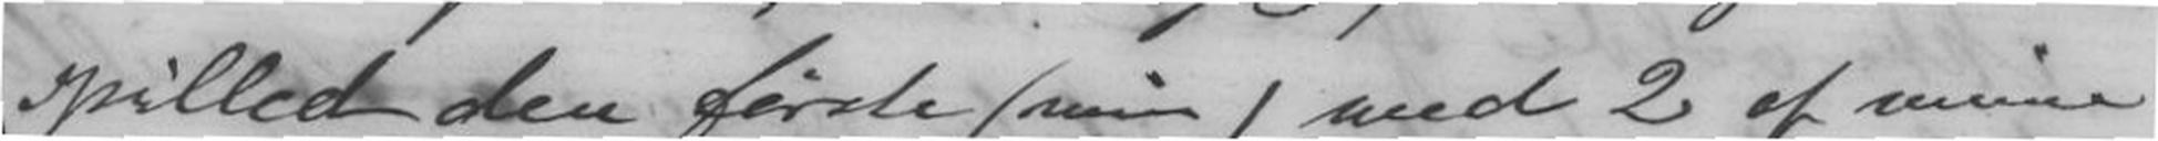spilled den første (min) med 2 af mine
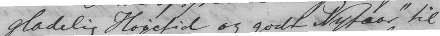glædelig Højetid og godt Nytaar" til
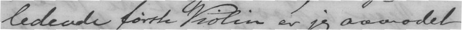ledende første Violin er jeg anmodet
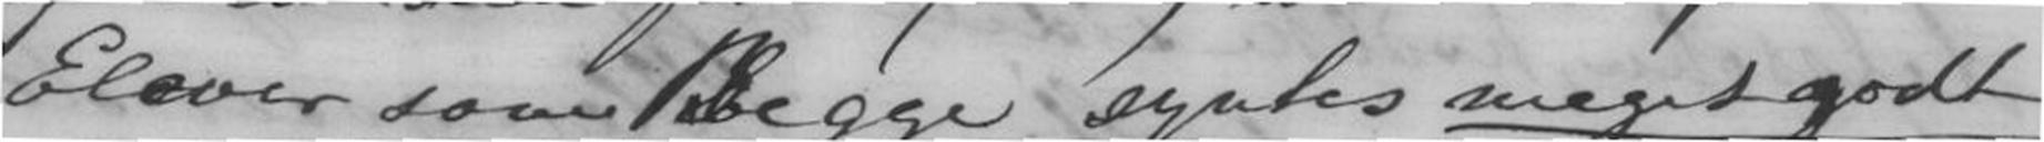Elever som Begge syntes meget godt
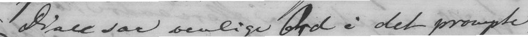De all saa venlige Ord i det promte
